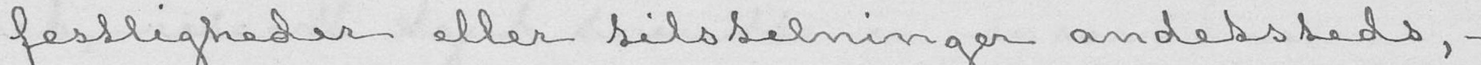festligheder eller tilstelninger andetsteds,-
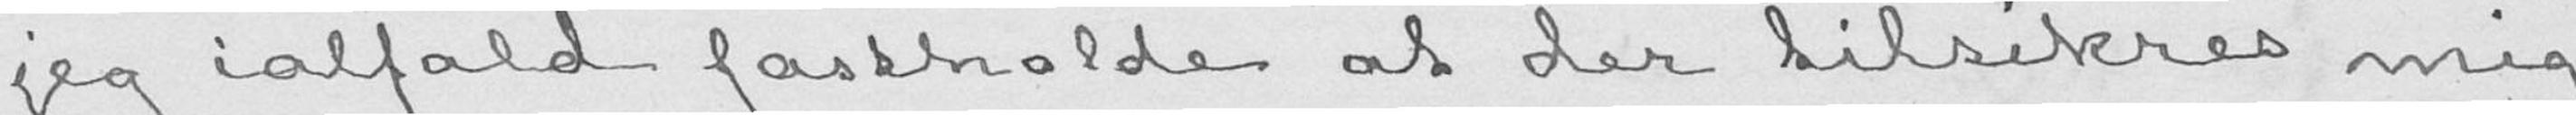jeg ialfald fastholde at der tilsikres mig
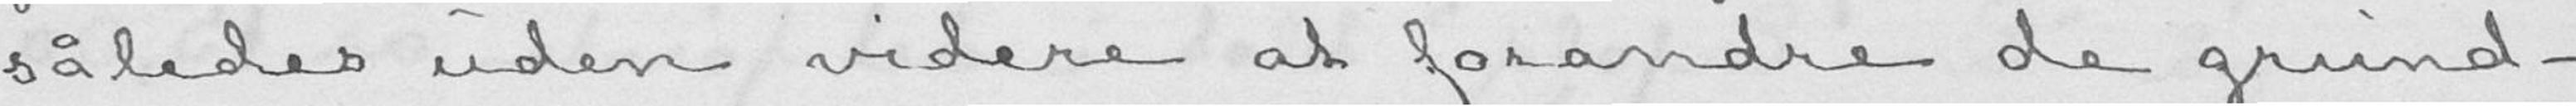således uden videre at forandre de grund-
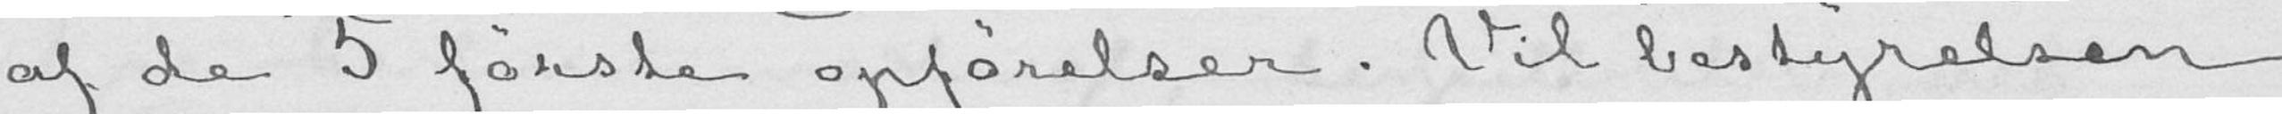af de 5 første opførelser. Vil bestyrelsen
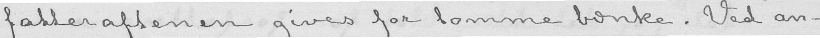fatteraftenen gives for tomme bænke. Ved an-
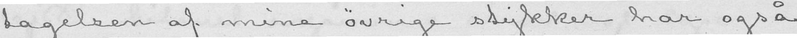tagelsen af mine øvrige stykker har også
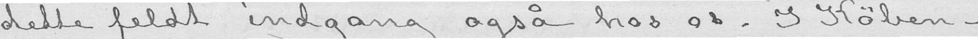dette feldt indgang også hos os. I Køben-
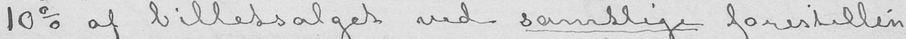10 % af billetsalget ved samtlige forestillin-
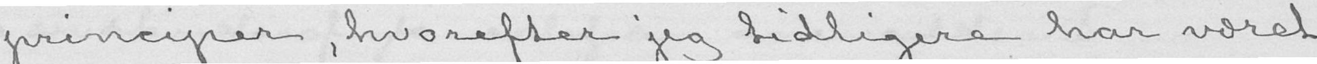principer, hvorefter jeg tidligere har været
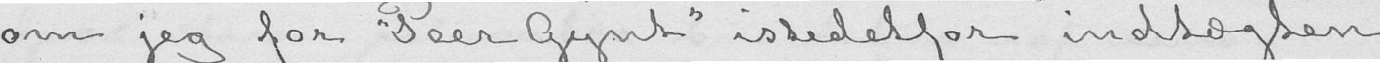om jeg for «Peer Gynt» istedetfor indtægten
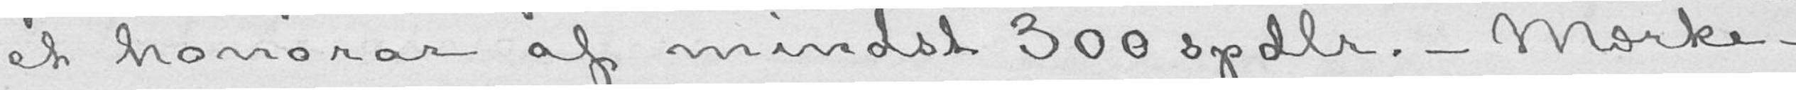et honorar af mindst 300 spdlr.- Mærke-
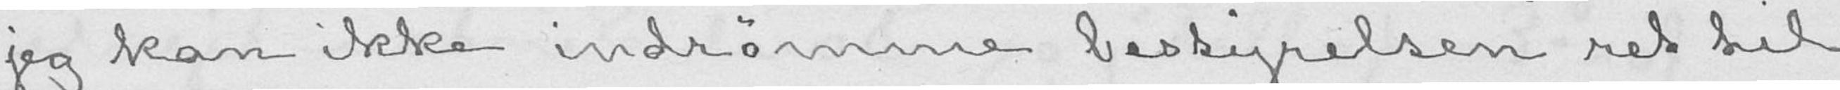jeg kan ikke indrømme bestyrelsen ret til
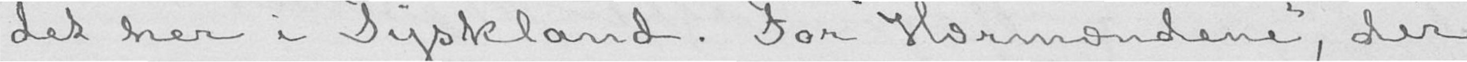det her i Tyskland. For «Hærmændene», der
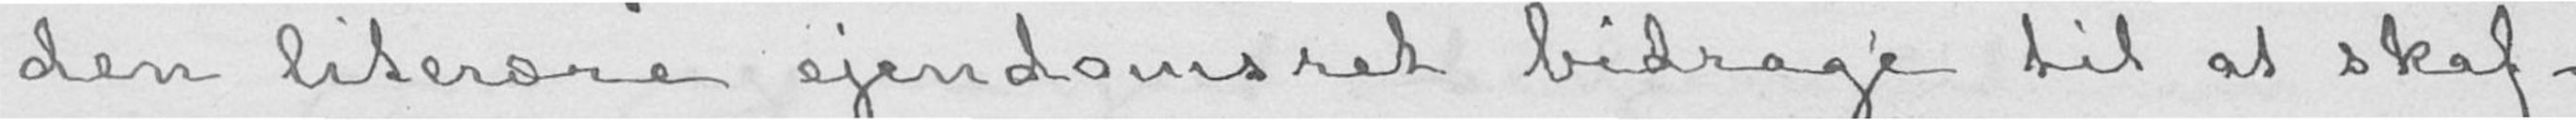den literære ejendomsret bidrage til at skaf-
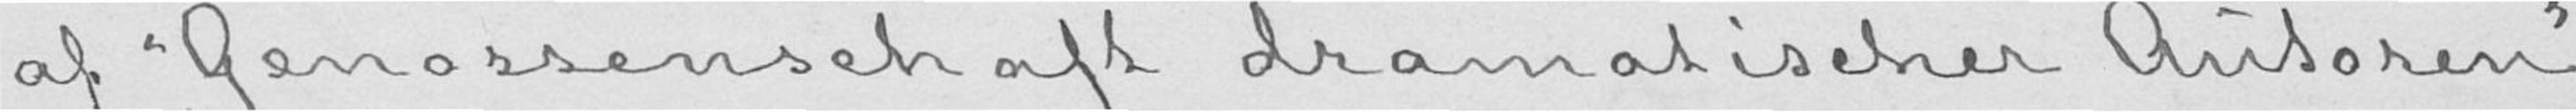af «Genossenschaft dramatischer Autoren»
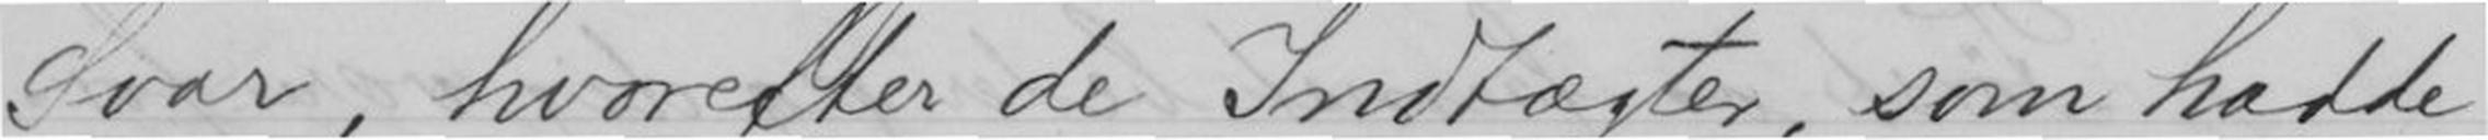Svar, hvorefter de Indtægter, som hadde
